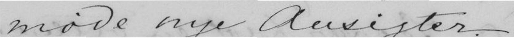møde nye Ansigter.
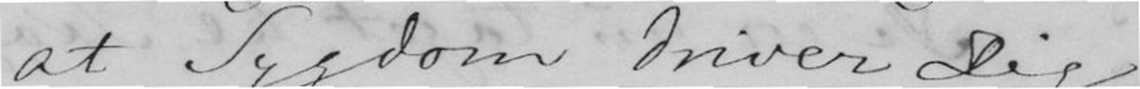at Sygdom driver dig
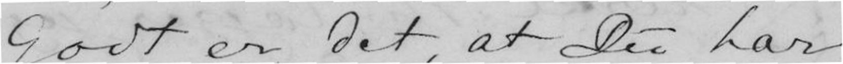Godt er det, at Du har
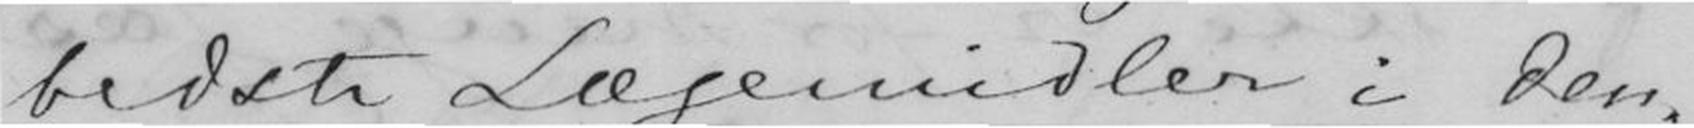bedste Lægemidler i den-
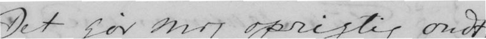Det gør mig oprigtig ondt,
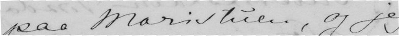paa Maristuen, og jeg
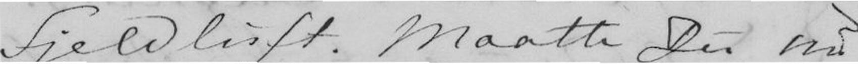Fjeldluft. Maatte Du nu
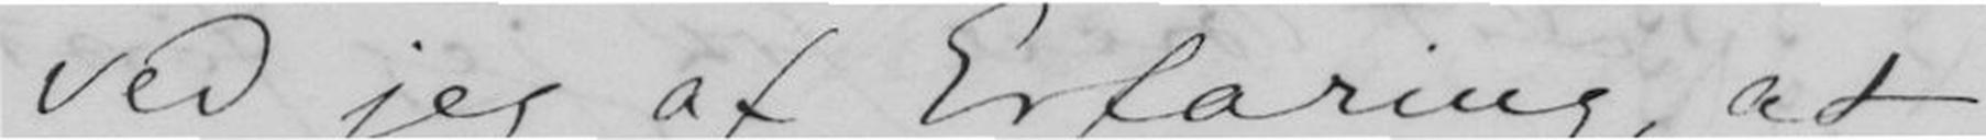ved jeg af Erfaring, at
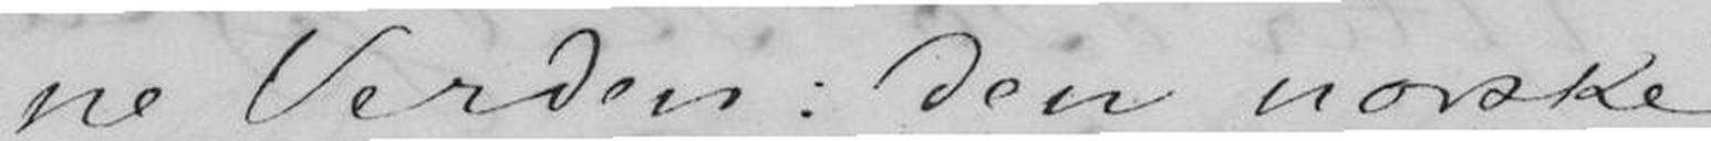ne Verden: Den norske
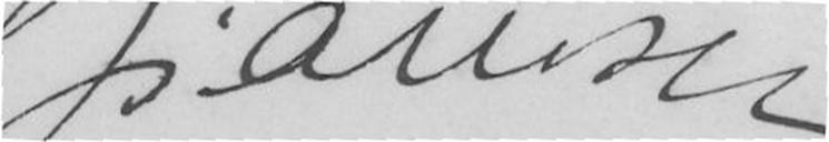B Bjørnson
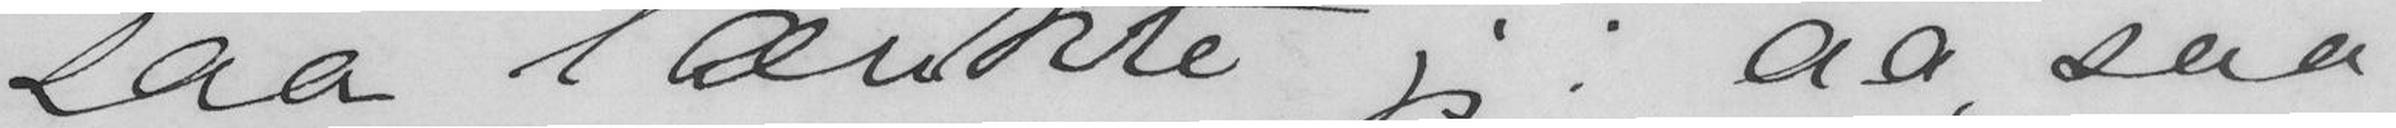saa tænkte jeg: aa, saa
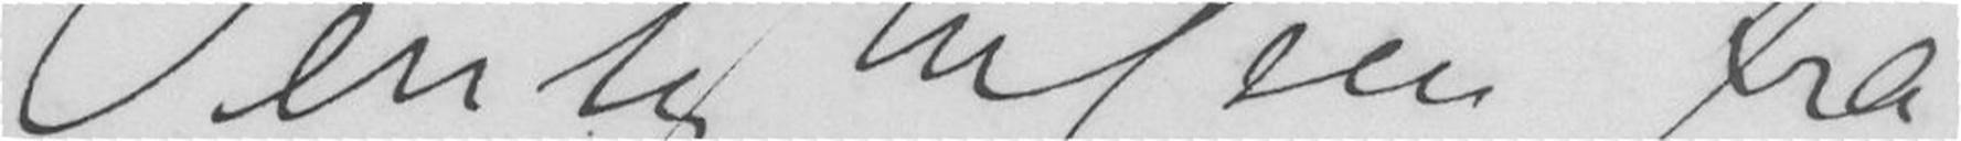Venlig hilsen fra
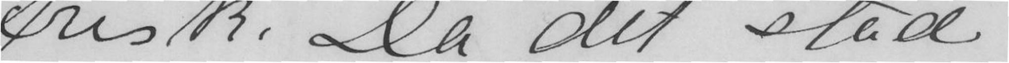frisk. Da det stod i
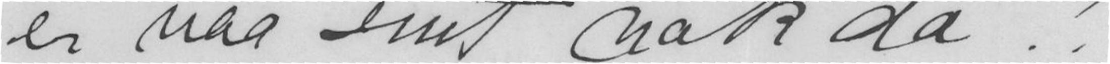er naa sint nok da!!
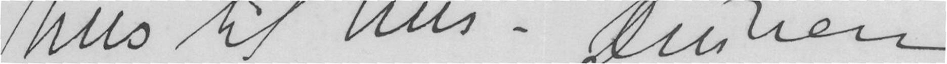hus til hus. Din ven
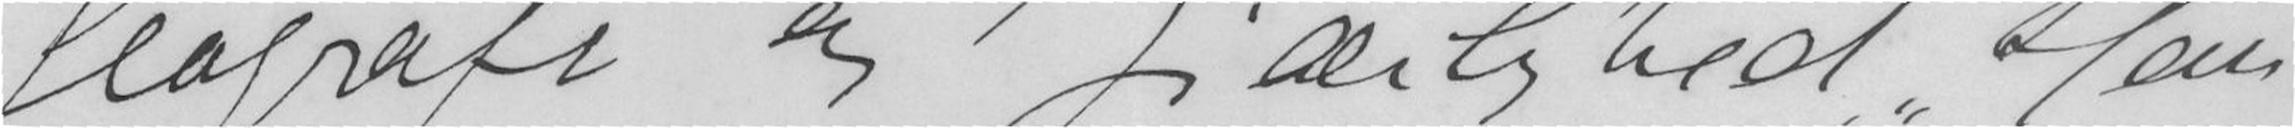Geografi og Kjærlighed, Han
